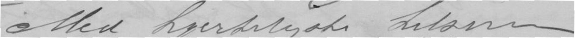Med hjerteligste hilsen
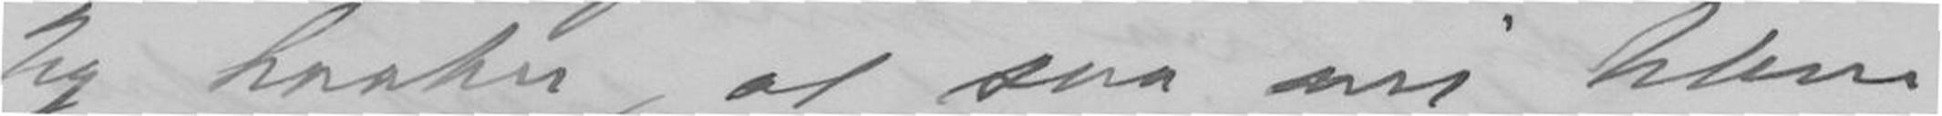Jeg haaber, at saa vil blive
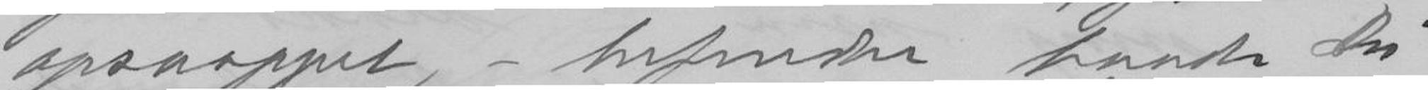opsnappet, - befinder baade Du
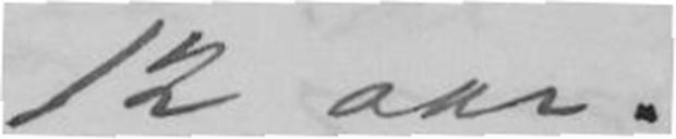12 aar.
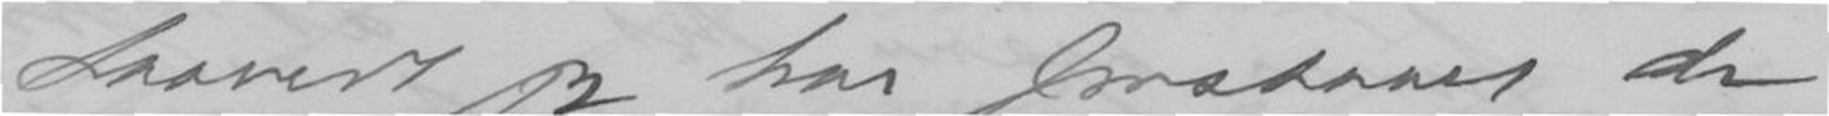Saavidt jeg har forstaaet de
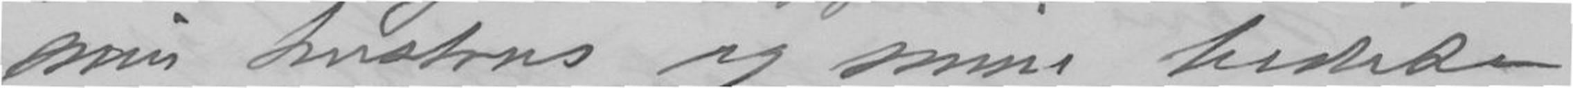min hustrus og mine bedste
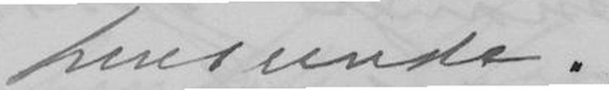henseende.
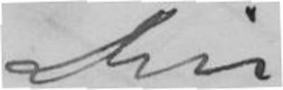Din
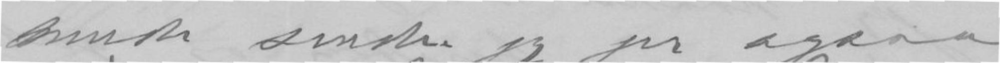mende sender jeg jer ogsaa
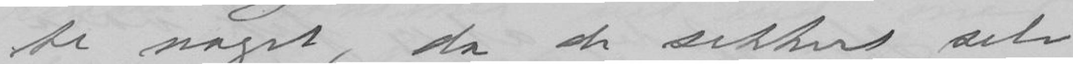te noget, da de sikkers selv
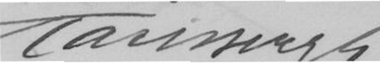Karl Bergh
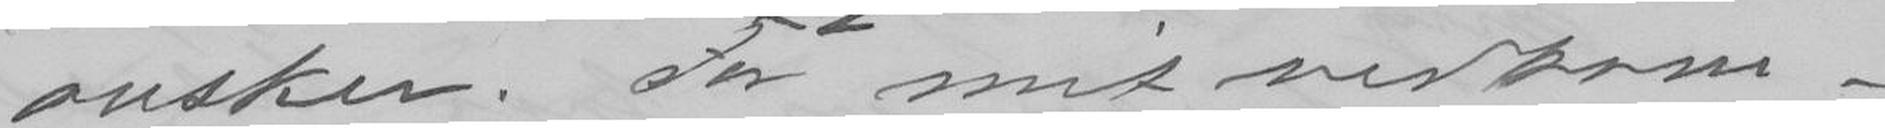ønsker. For mit vedkom-
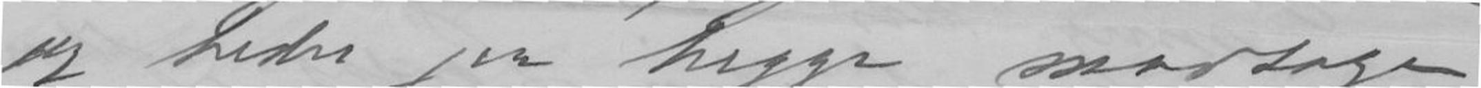jeg beder jer begge modtage
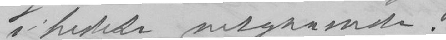i bedste velgaaende.
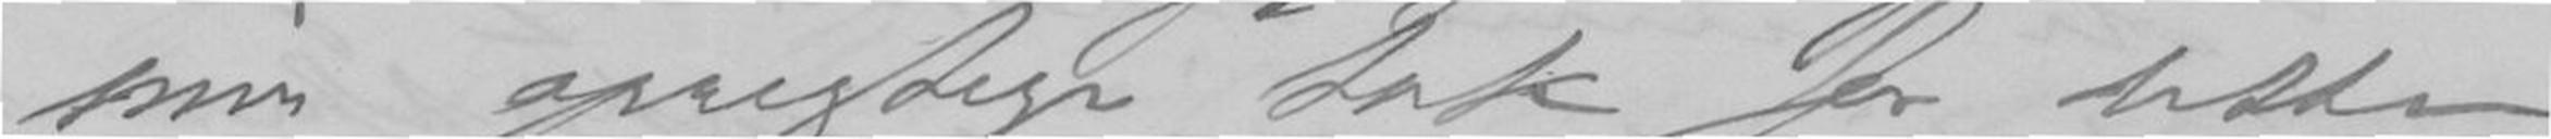min oprigtige tak for dette
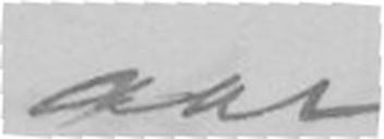aar.
